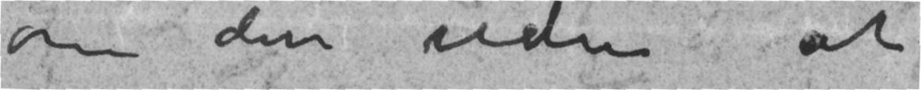om den uden at
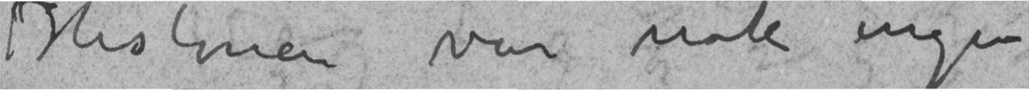Historien var nok ingen
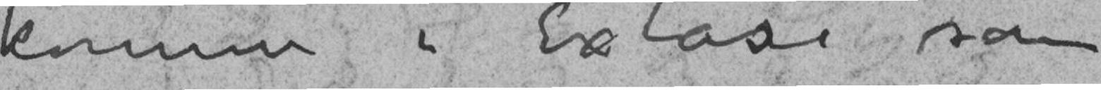komme i Extase som
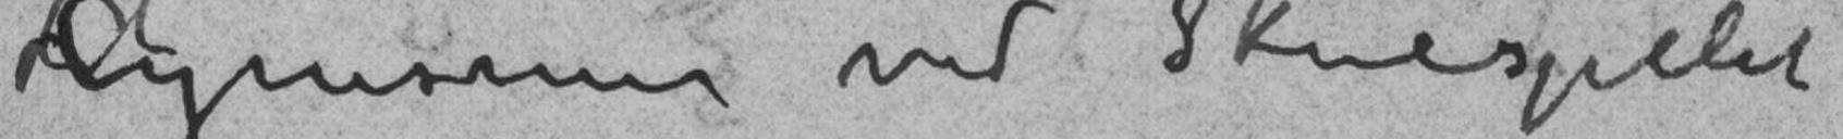detCynismen ved Skuespillet
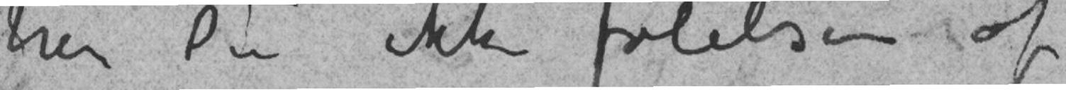har Du ikke Følelsen af
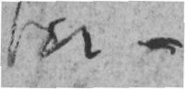før –
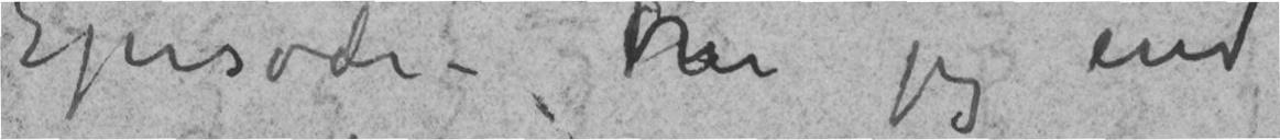Episode – Om jeg end
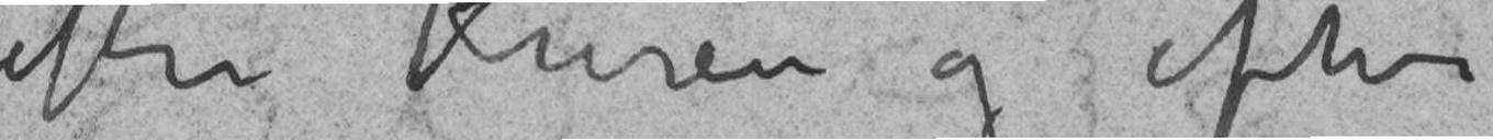efter Kuren og efter
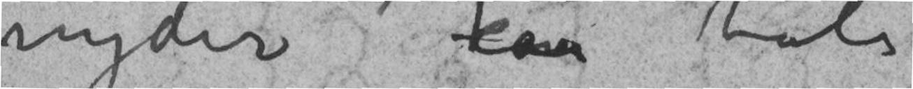nyder kan tale
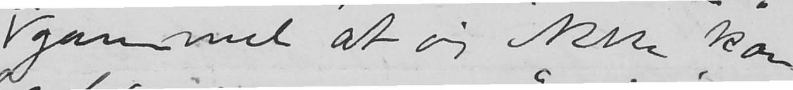gammel at jeg ikke kan
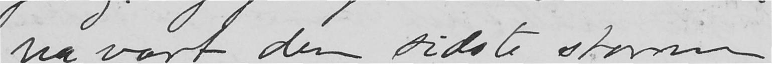ha vært den sidste storm
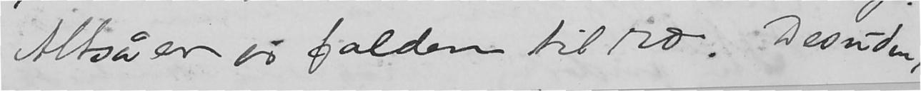Altså er jeg falden til ro. Desuden,
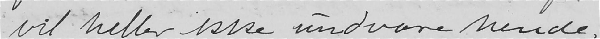vil heller ikke undvære hende,
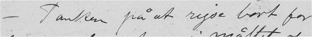- Tanken på at rejse bort for
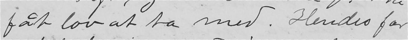fåt lov at ta med. Hendes far
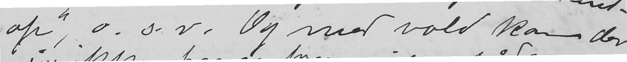op", o.s.v. Og med vold kan der
